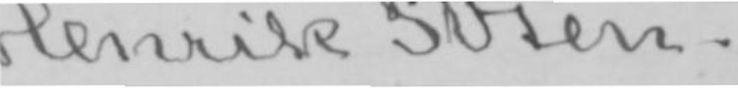Henrik Ibsen.
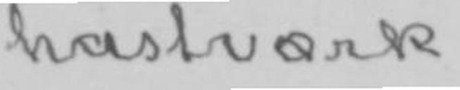hastværk
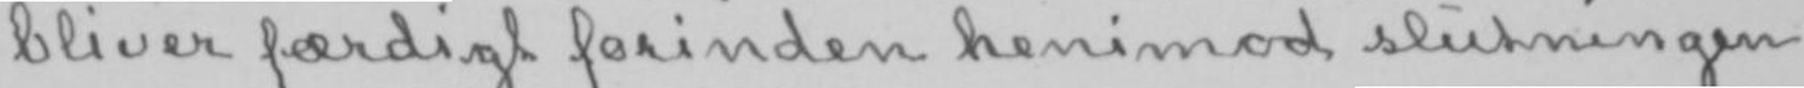bliver færdigt forinden henimod slutningen
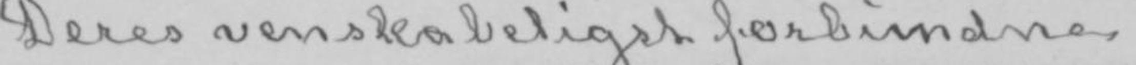Deres venskabeligst forbundne
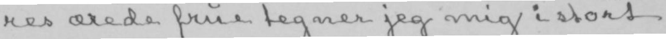res ærede frue tegner jeg mig i stort
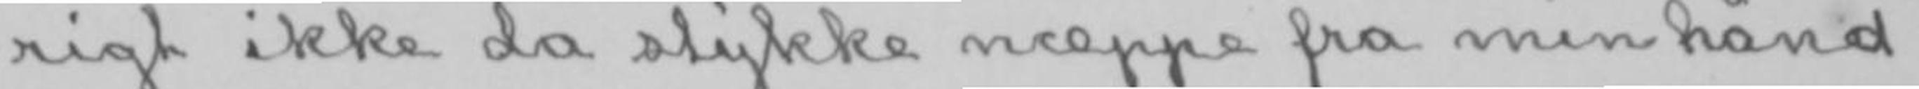rigt ikke da stykke næppe fra min hånd
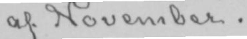af November.
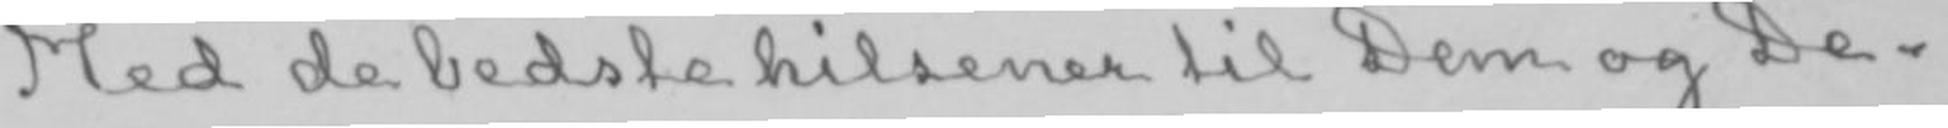Med de bedste hilsener til Dem og De-
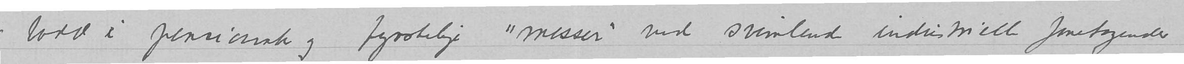bodd i pensionat og fyrstelige «messer»  ved svimlende industrielle foretagender
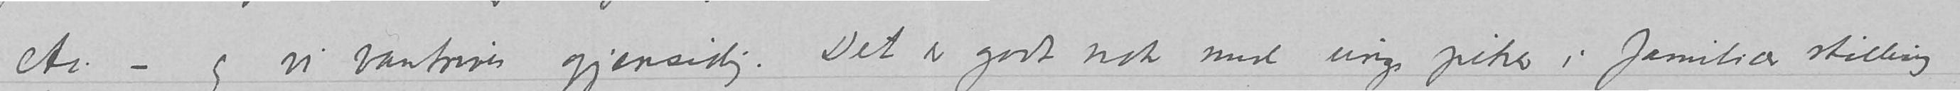etc. – og vi vantrives gjensidig. Det er godt nok med unge piker i familier stilling
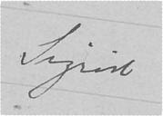Sigrid.
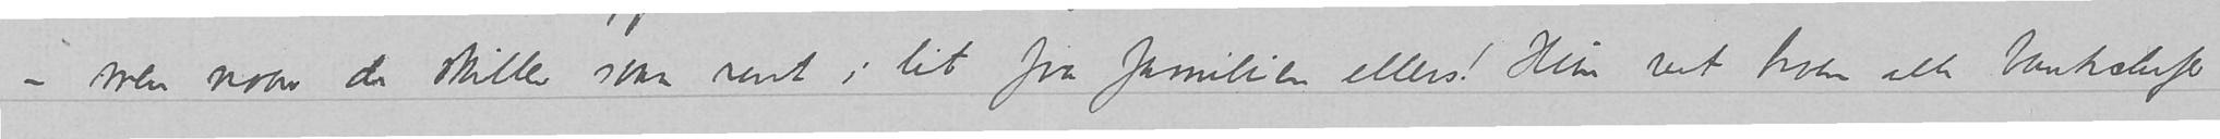–men naar de skiller sam rent i lit fra familien ellers! Hun vet hvem alle bankchefer
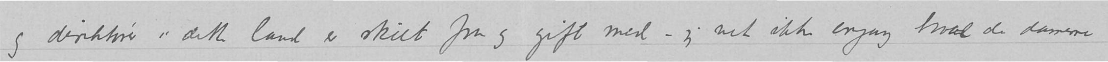og direktører i dette land er skilt fra og gift med jeg vet ikke engang hvad de damerne
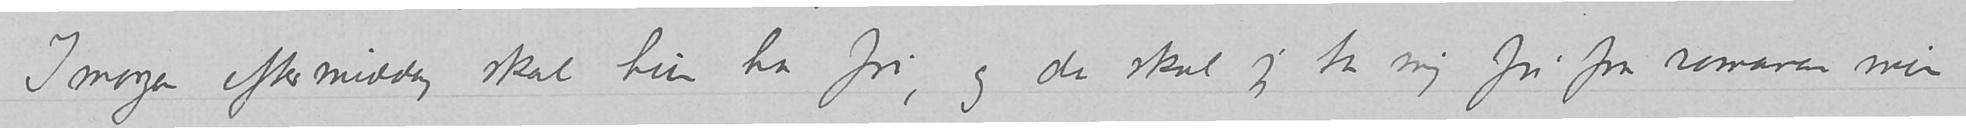Imorgen eftermiddag skal hun har fri, og da skal jeg ta mig fri fra romanen min
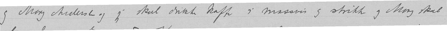og Mary Andersen og jeg skal drikke kaffe i massvis og strikke og Mary skal
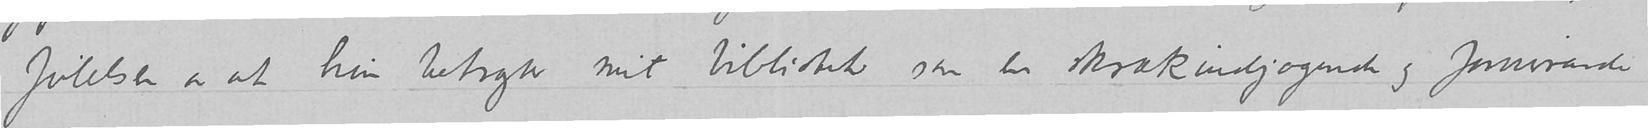følelsen av at hun betragter mit bibliotek som en skrækindjagende og forvirrende
